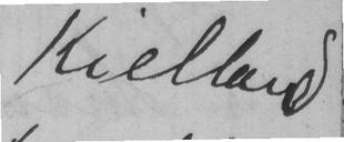Kielland
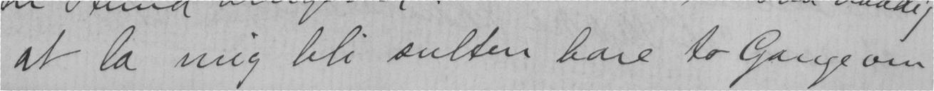at la mig bli sulten bare to Gange om
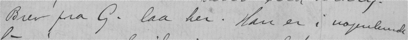Brev fra G. laa her. Han er i nogenlunde
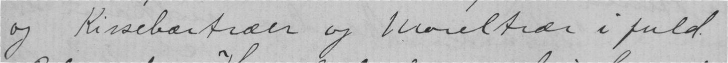og Kirsebærtræer og Moreltrær i fuld
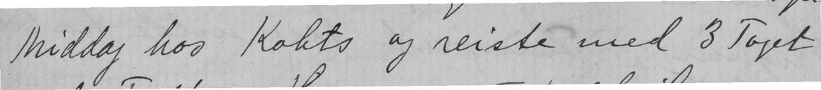Middag hos Kohts og reiste med 3 Toget
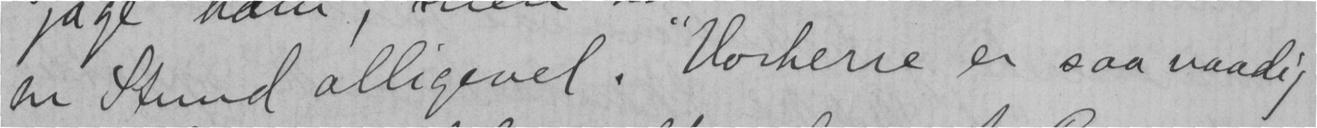en Stund alligevel. "Vorherre er saa naadig
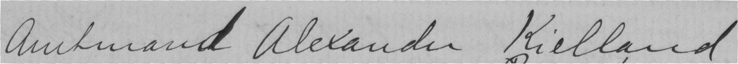Amtmand Alexander Kielland
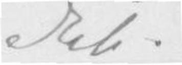Feb.
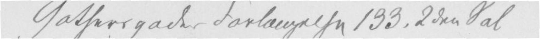Gothersgades Forlængelse 133, 2den Sal
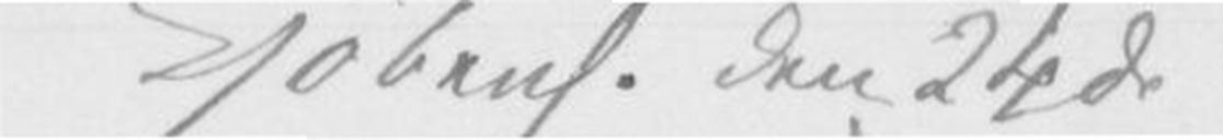Kjøbenh. Den 24de
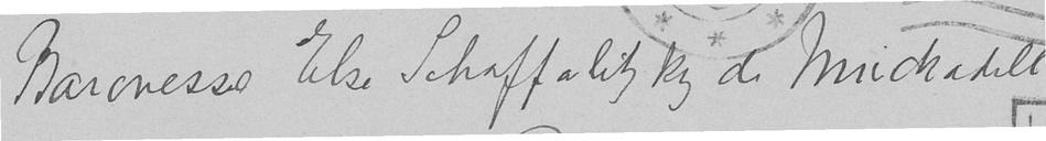Baronesse Else Schaffalitzky de Muckadell
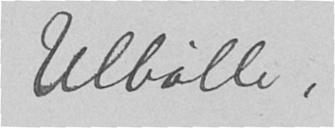Ulbølle.
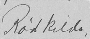Rødkilde,
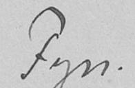Fyn.
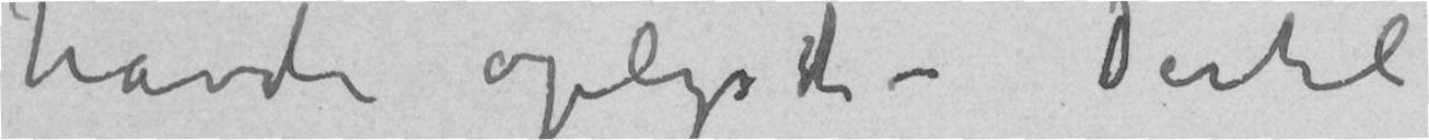havde oplyst – Dertil
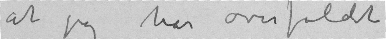at jeg har overfaldt
